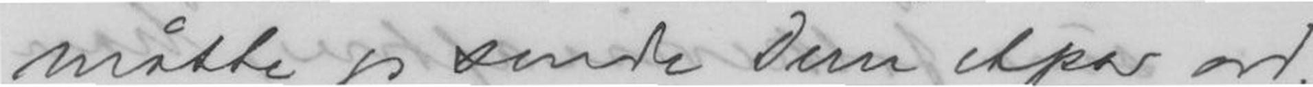måtte jeg sende Dem etpar ord,
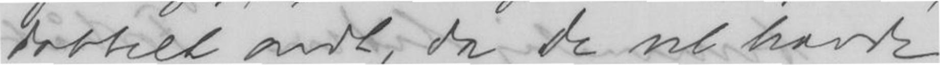dobbelt ondt, da de vil havde
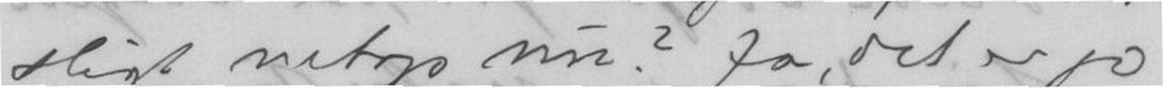sligt netop nu? Ja, det er jo
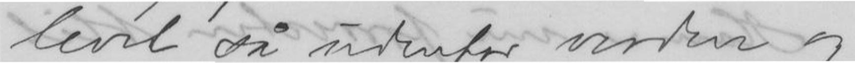levet så udenfor verden og
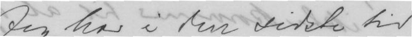Jeg har i den sidste tid
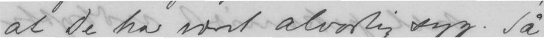at De har vært alvorlig syg. Så
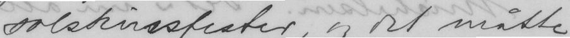solskinsfester, og det måtte
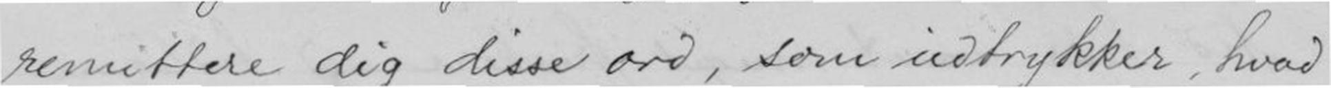remittere dig disse ord, som udtrykker hvad
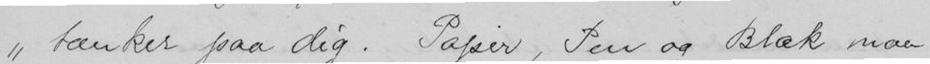"tænker paa dig. Papir, Pen og Blæk maa
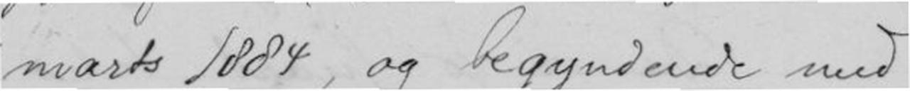marts 1884, og begyndende med
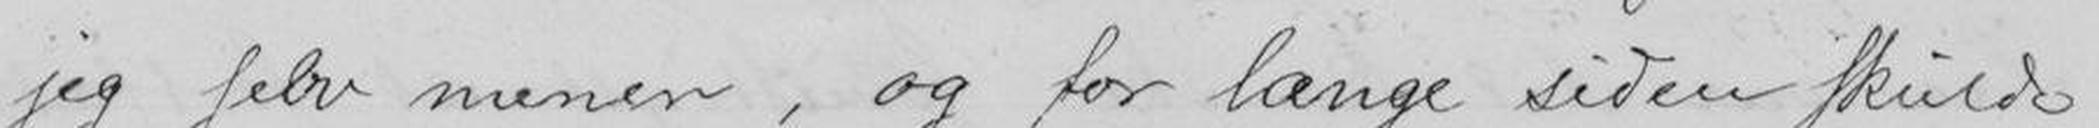jeg selv mener, og for længe siden skulde
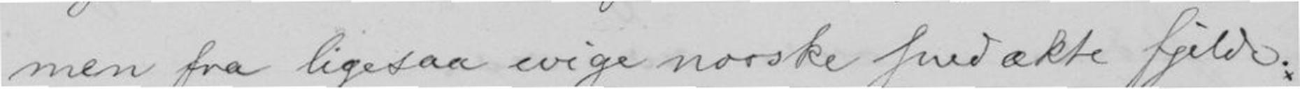men fra ligesaa evige norske fuldækte fjelde.
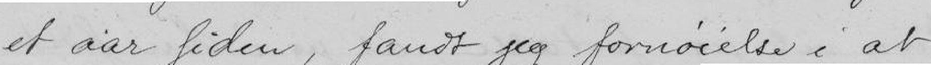et aar siden, fandt jeg fornøielse i at
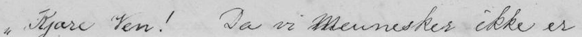"Kjære Ven! Da vi Mennesker ikke er
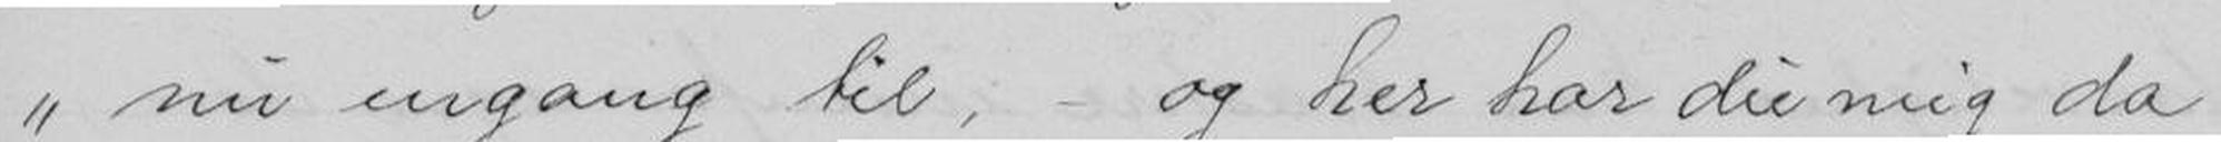"nu engang til; - og her har du mig da
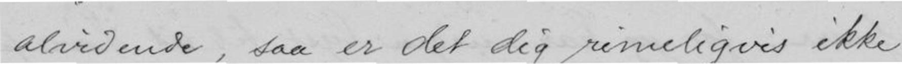"alvidende, saa er det dig rimeligvis ikke
